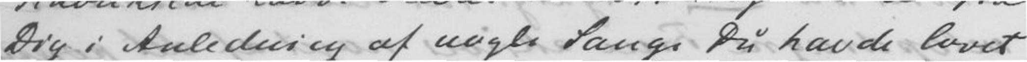Dig i Anledning af nogle Sange Du havde lovet
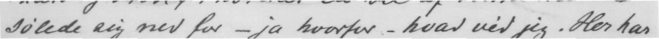sølede sig ned for - ja hvorfor - hvad vèd jeg. Her har
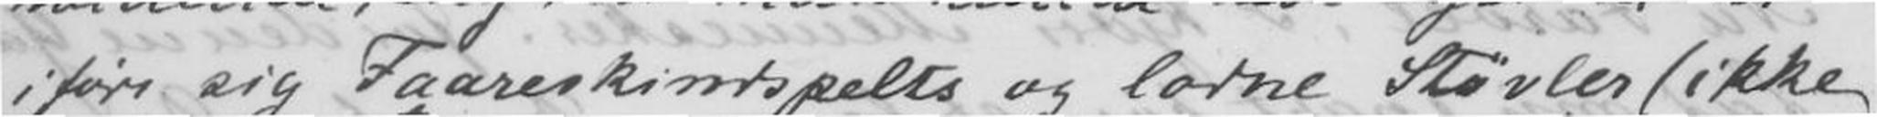iføre sig Faareskindspelts og lodne Støvler (ikke
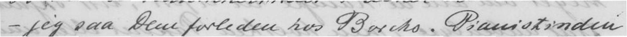- jeg saa Dem forleden hos Boschs. Pianistinden
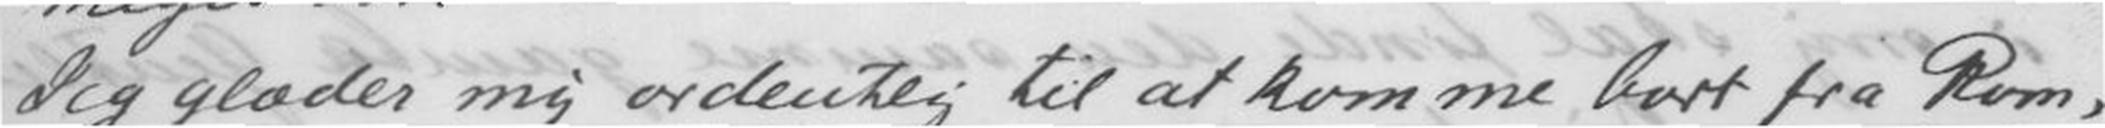Jeg glæder mig ordentlig til at komme bort fra Rom,
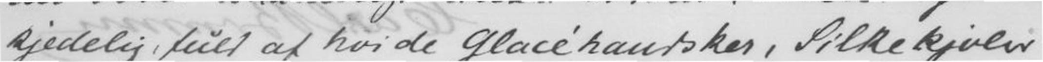kjedelig fuldt af hvide Glacèhandsker, Silkekjoler
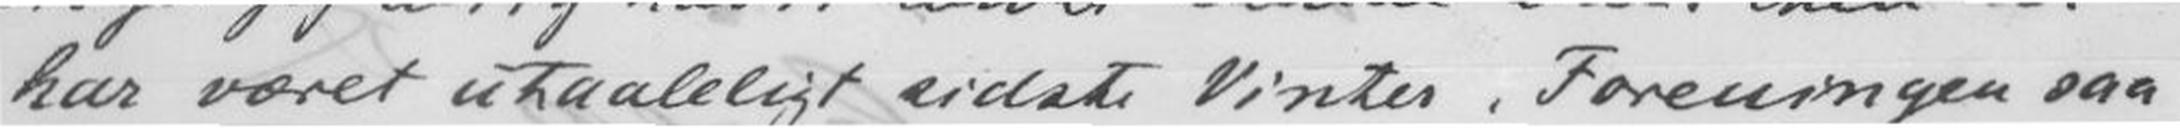har været utaalelig sidste Vinter. Foreningen saa
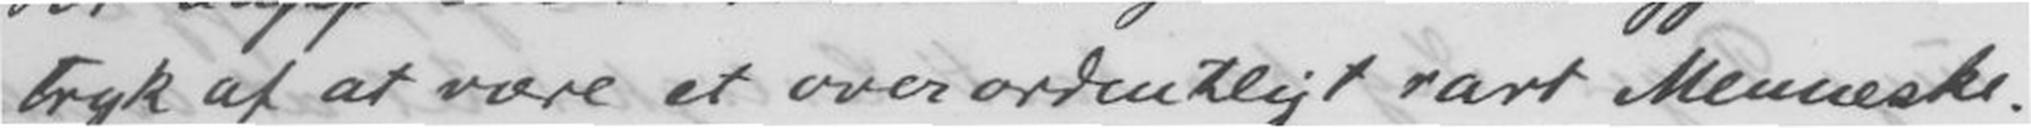tryk af at være et overordentlig rart Menneske.
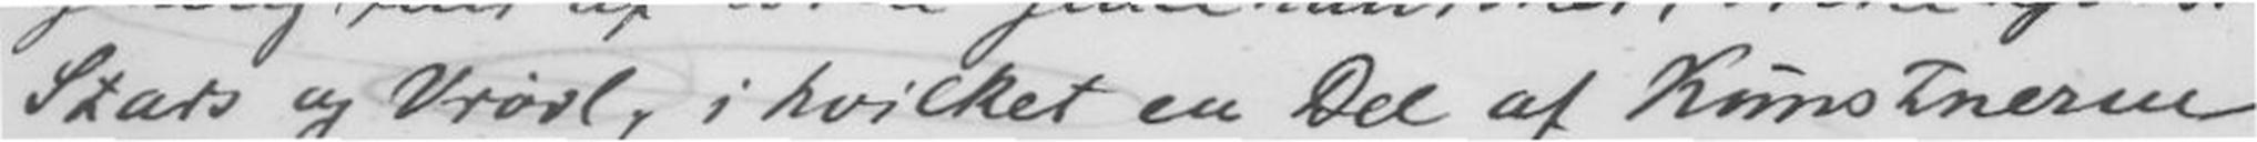Stads og Vrøvl, i hvilket en Del af Kunstnerne
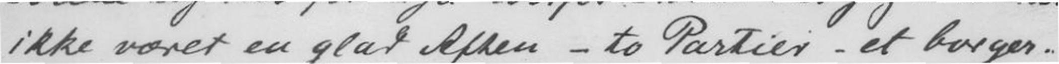ikke været en glad Aften - to Partier - et borger-
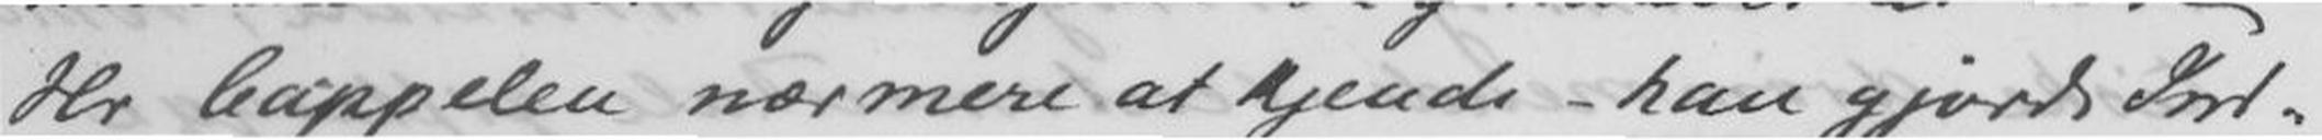Hr Cappelen nærmere at kjende - han gjorde Ind-
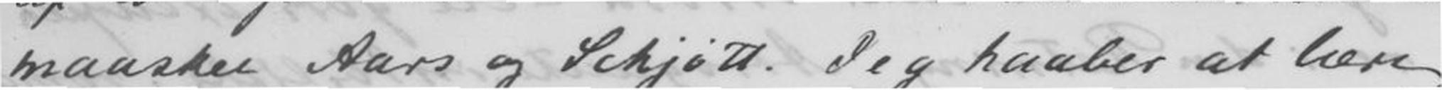maaskee Aars og Schjøtt. Jeg haaber at lære
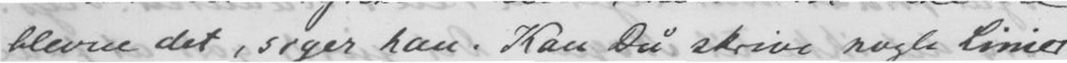bleven det, siger han. Kan Du skrive nogle Linier
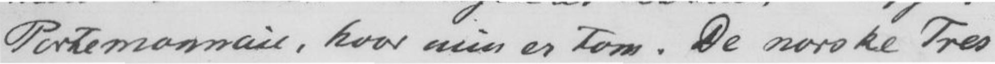Portemonnaie, hvor min er Tom. De norske Tres-
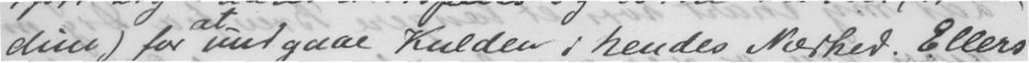dine) for undgaae Kulden i hendes Nærhed. Ellers
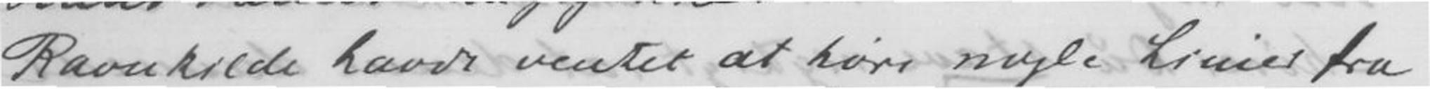Ravnkilde havde ventet at høre nogle Linier fra
